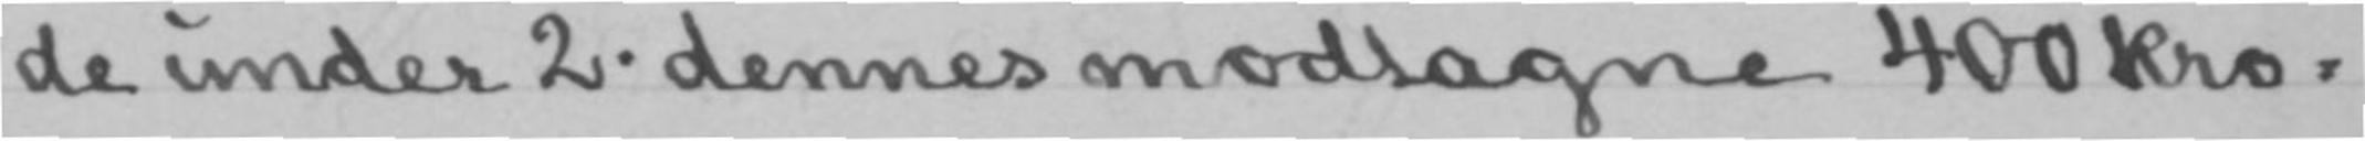de under 2. dennes modtagne 400 kro-
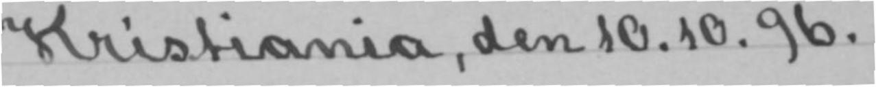Kristiania, den 10.10.96.
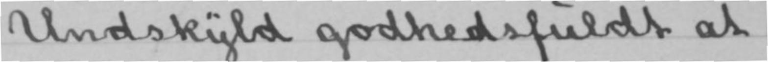Undskyld godhedsfuldt at
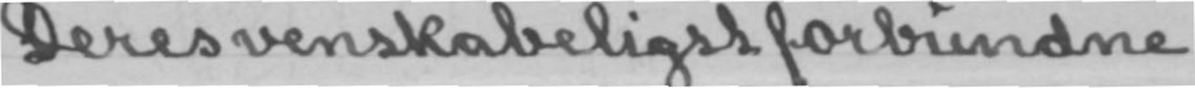Deres venskabeligst forbundne
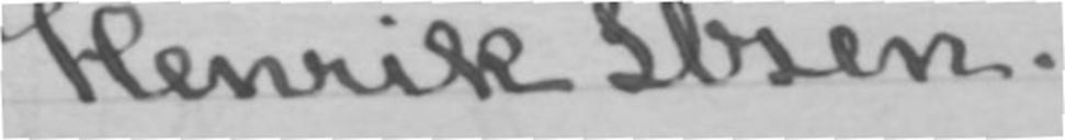Henrik Ibsen.
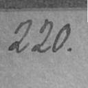220.
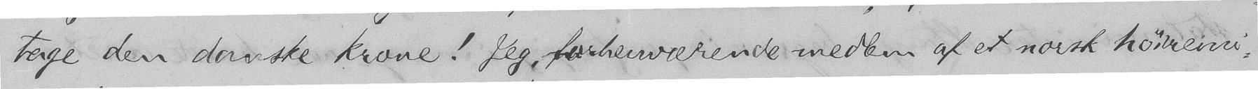tage den danske krone! Jeg, forhenværende medlem af et norsk høiremi-
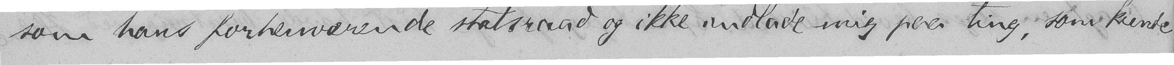som hans forhenværende statsraad og ikke indlade mig paa ting, som kunde
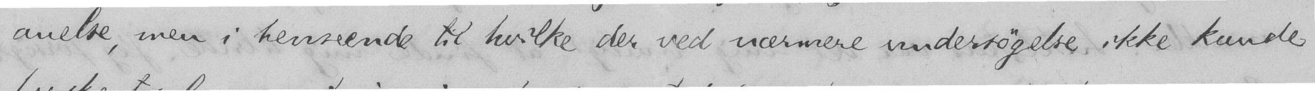anelse, men i henseende til hvilke der ved nærmere undersøgelse ikke kunde
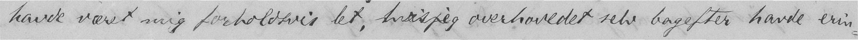havde været mig forholdsvis let, hvis jeg overhovedet selv bagefter havde erin-
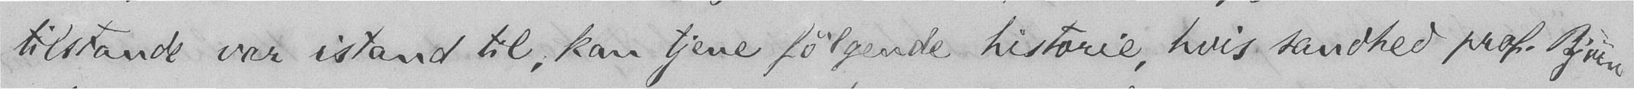tilstande var istand til, kan tjene følgende historie, hvis sandhed prof. Björn-
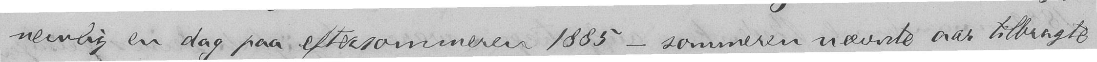nemlig en dag paa eftersommeren 1885 - sommeren nævnte aar tilbragte
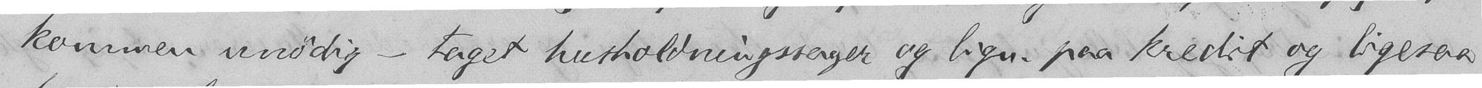kommen unødig - taget husholdningssager og lign. paa kredit og ligesaa
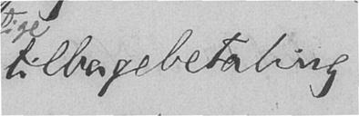tilbagebetaling
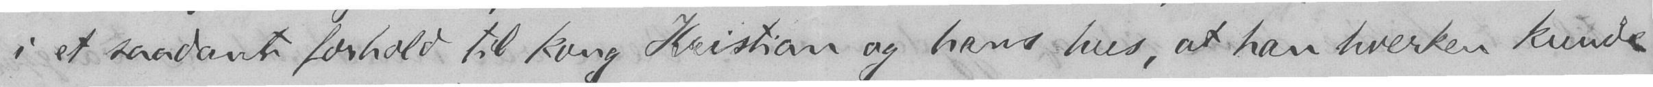i et saadant forhold til kong Kristian og hans hus, at han hverken kunde
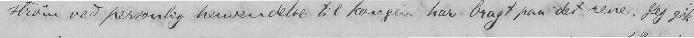ström ved personlig henvendelse til kongen har bragt paa det rene. Jeg gik
